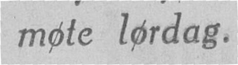møte lørdag.
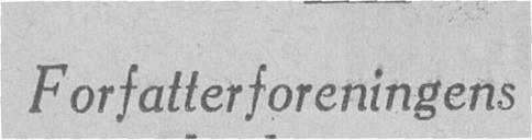Forfatterforeningens
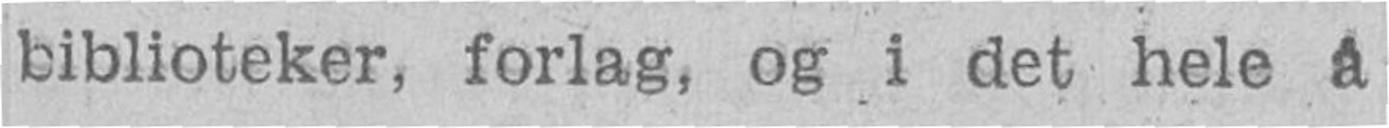biblioteker, forlag, og i det hele å
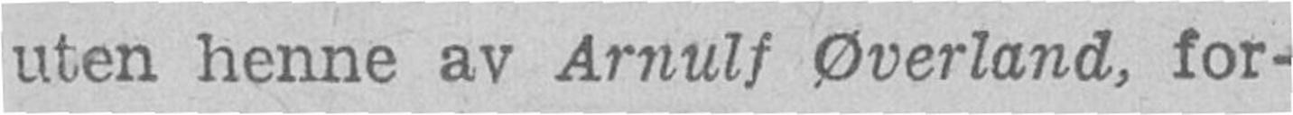uten henne av Arnulf Øverland, for-
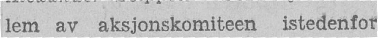lem av aksjonskomiteen istedenfor
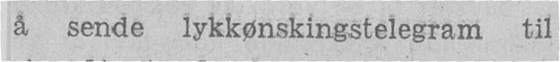å sende lykkønskingstelegram til
